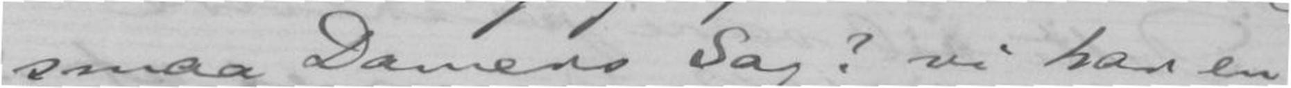smaa Damers Sag? vi har en
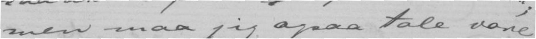men maa jeg ogsaa tale vore
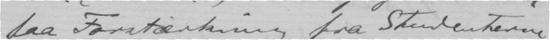faa Forstærkning fra Studenterne
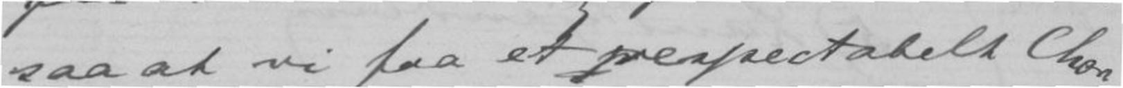saa at vi faa et respectabelt Chor;
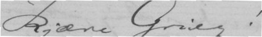Kjære Grieg!
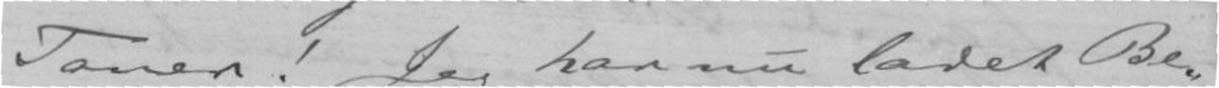Toner!. Jeg har nu ladet Be-
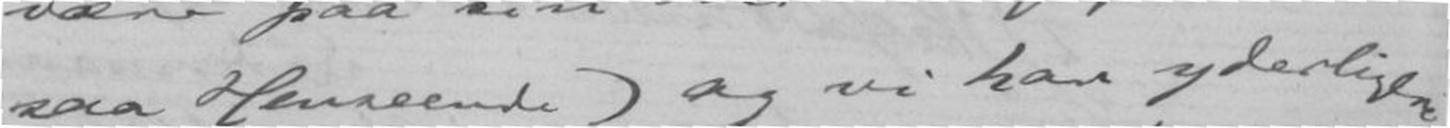saa Henseende ) og vi har yderligere
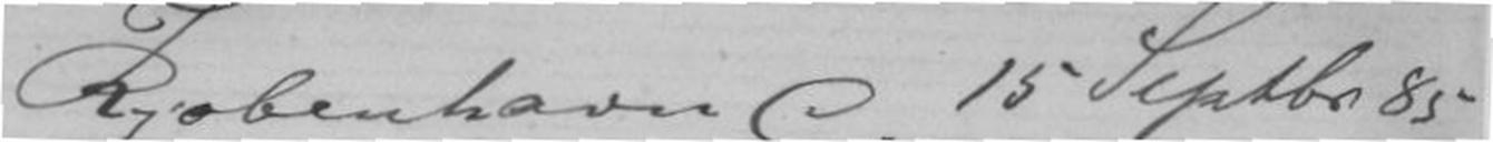Kjøbenhavn d. 15 Septbr 85
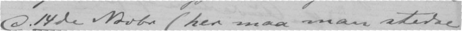d. 14de Novbr. (her maa man stedse
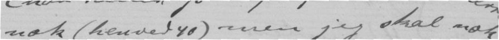nok (henved 40) men jeg skal nok
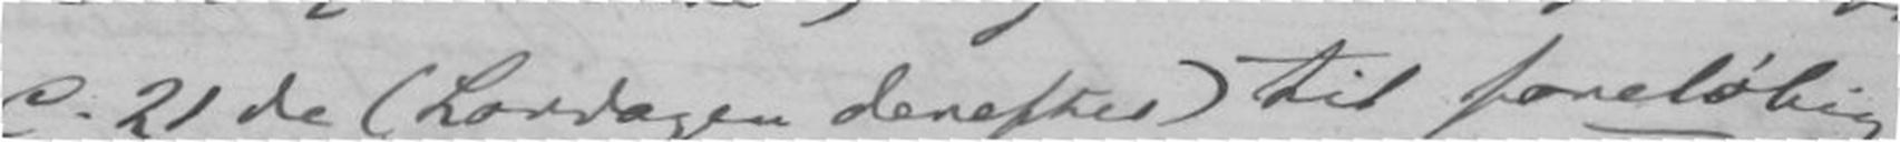d. 21de (Lørdagen derefter) til foreløbig
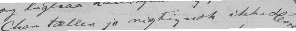Chor tæller jo rigtignok ikke Herrer
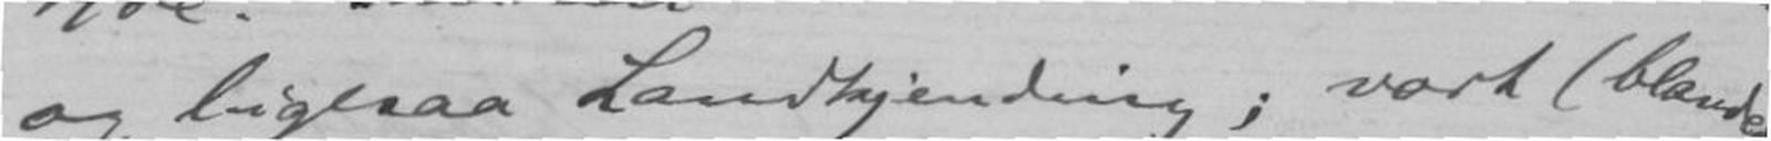og ligesaa Landkjending; vort (blandede)
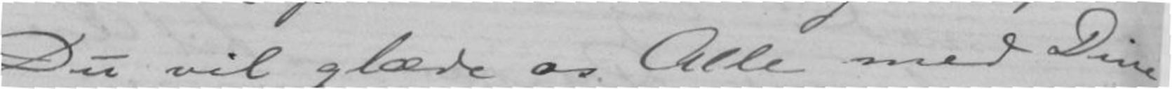Du vil glæde os Alle med Dine
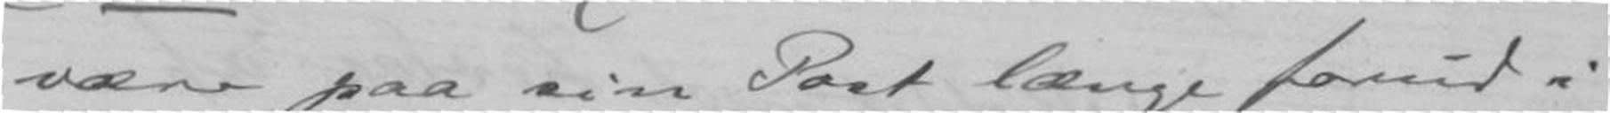være paa sin Post længe forud i
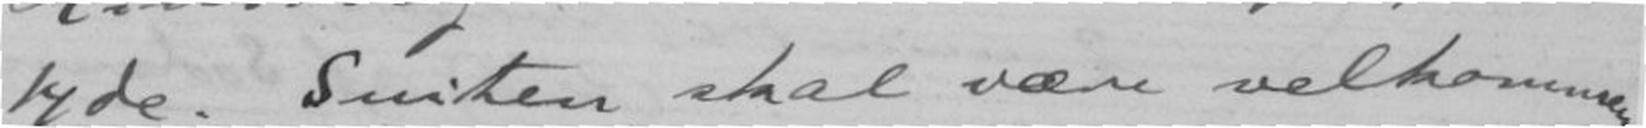14de. Suiten skal være velkommen
